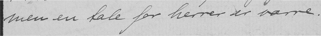men en tale for herrer er værre.
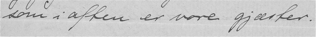som i aften er vore gjæster.
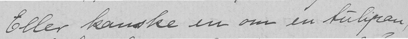Eller kanske en om en tulipan,
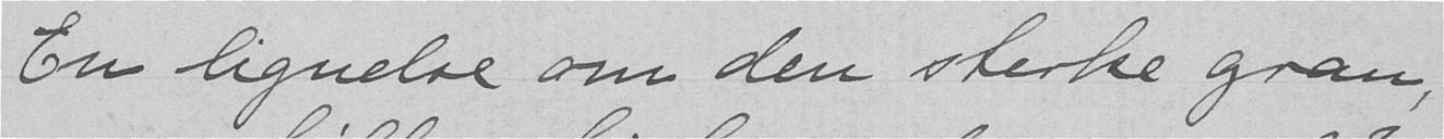En lignelse om den sterke gran,
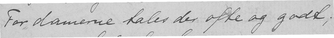For damerne tales der ofte og godt;
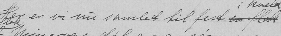Her er vi nu samlet til fest en flok
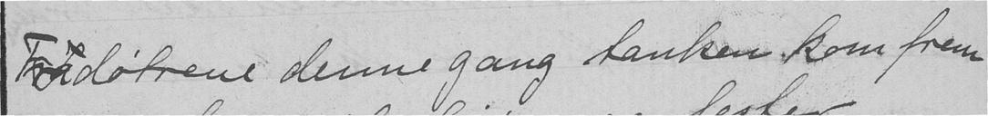Fra døtrene denne gang tanken kom frem
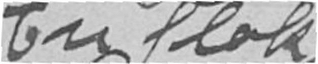En flok
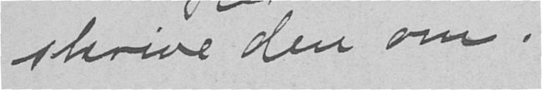skrive den om.
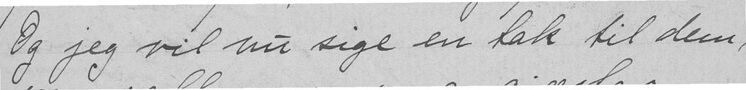Og jeg vil nu sige en tak til dem,
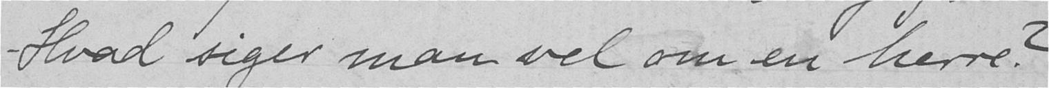- Hvad siger man vel om en herre?
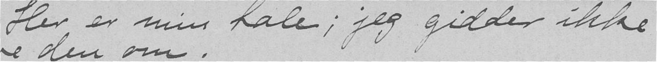Her er min tale; jeg gidder ikke
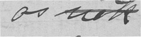os nok
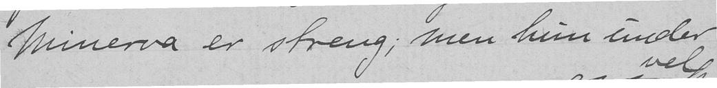Minerva er streng; men hun under
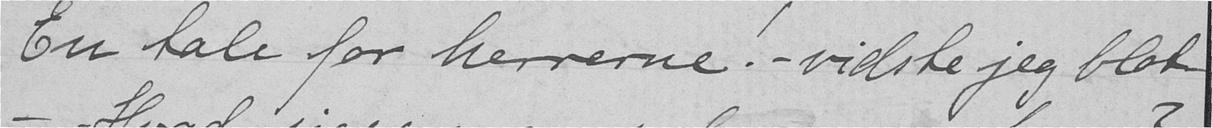En tale for herrerne! - vidste jeg blot
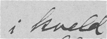i kveld
